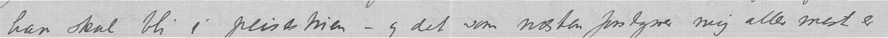han skal bli i peisestuen – og det som næsten forstyrrer mig aller mest er
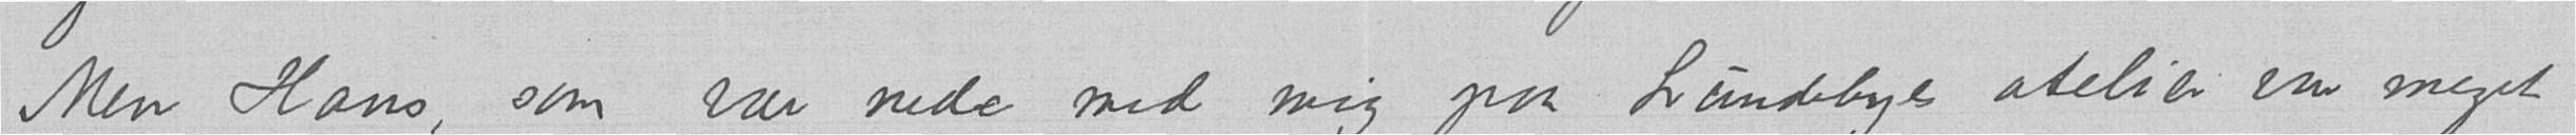Men Hans, som var nede med mig paa Lundebyes atelier var meget
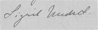Sigrid Undset.
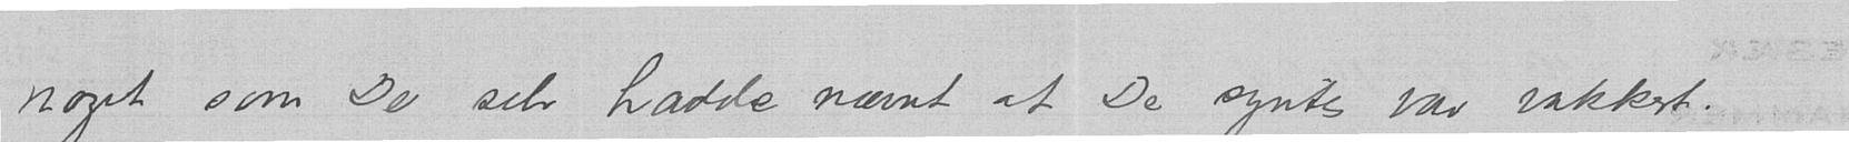noget som De selv hadde nævnt at De syntes var vakkert.
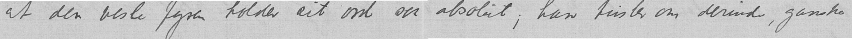at den vesle fyren holder sit ord saa absolut; han tusler om derinde, ganske
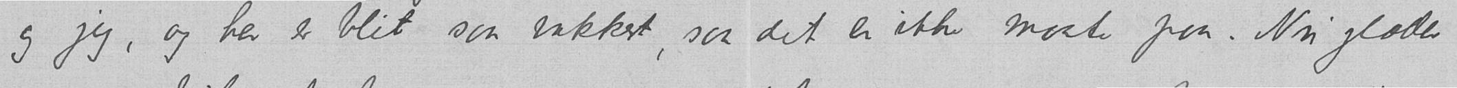og jeg, og her er blit saa vakkert saa det er ikke maate paa. Nu glæder
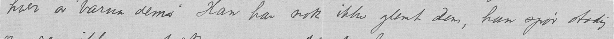hver av "barna dems"  Han har nok ikke glemt dem, han spør stadig
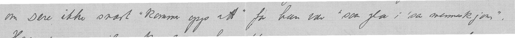om Dere ikke snart "kommer opp att» for han var "saa gla i 'sa menneskjom".
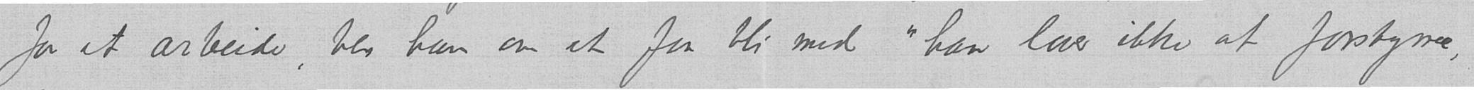for at arbeide, ber han om at faa bli med "han lover ikke at forstyrre,
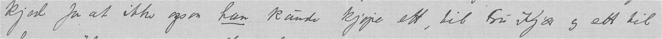kjæd for at ikke ogsaa han kunde kjøpe ett, til Fru Kjær og ett til
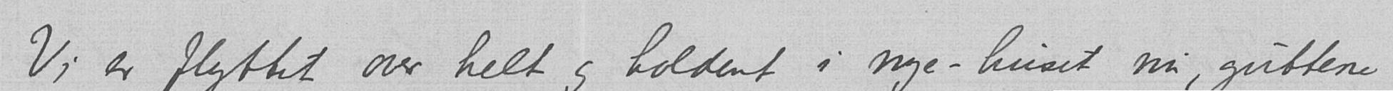Vi er flyttet over helt og holdent i nye-huset nu, guttene
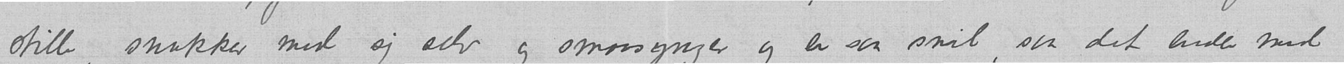stille, snakker med sig selv og smaasynger og er saa snil, saa det ender med
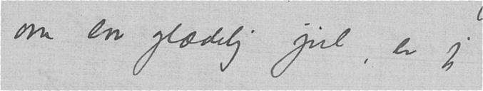om en glædelig jul, er jeg
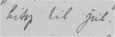hitop til jul.
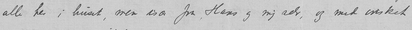alle her i huset, men især fra Hans og mig selv, og med ønsket
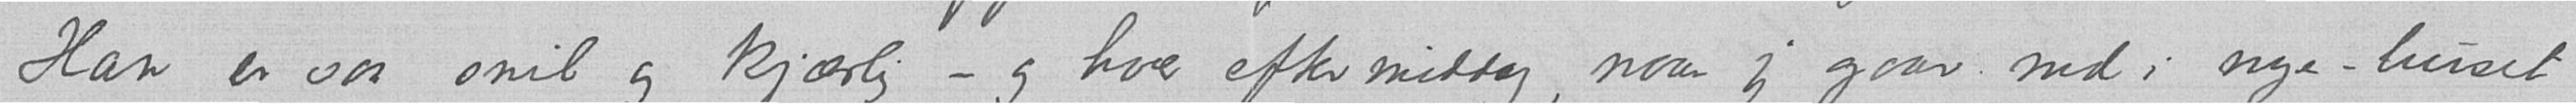Han er saa snil og kjærlig – og hver eftermiddag, naar jeg gaar ned i nye-huset
